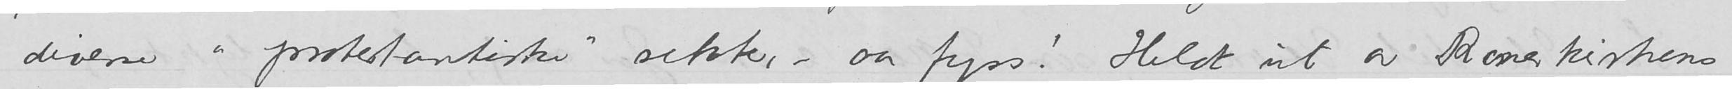diverse i «protestantiske» sekter – aa fyss! Heldt ut av Romerkirkens
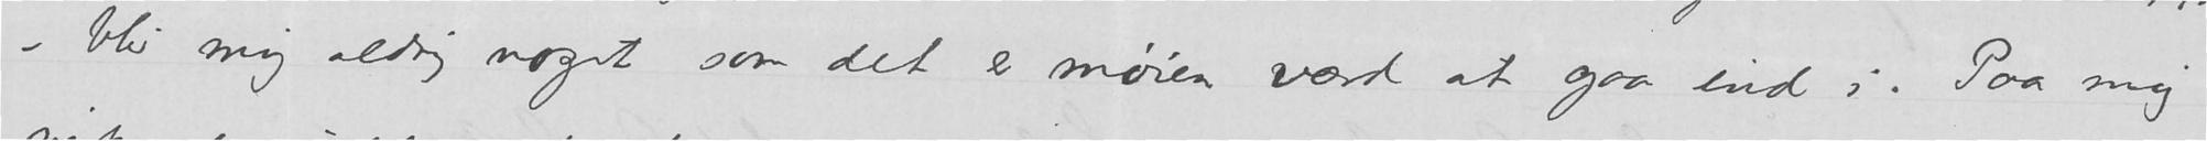– blir mig aldrig noget som det er møien værd at gaa ind i. Paa mig
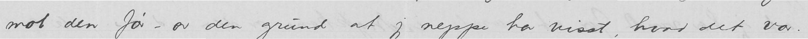mot den før – av den grund at jeg neppe har visst, hvad det var.
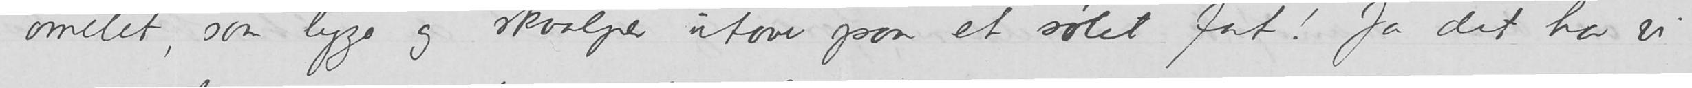omelet, som ligger og skvalper utover paa et sølet fat! Ja det har vi
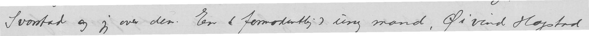Svarstad og jeg over den. En (formodentlig) ung mand, Øivind Hogstad
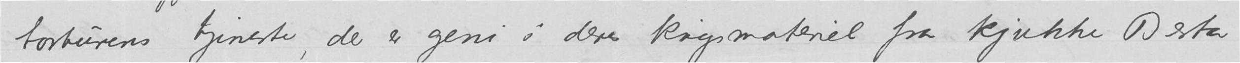torturens tjeneste der er geni i deres krigsmateriel fra kjukke Berta
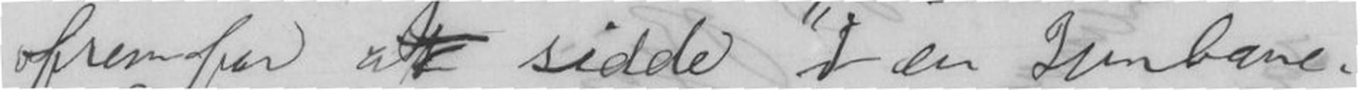fremfor at sidde i en Jernbane-
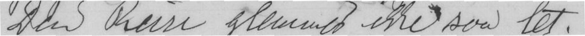Den Reise glemmes ikke saa let.
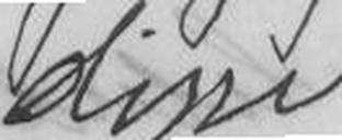disse
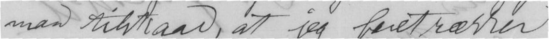maa tilstaae, at jeg foretrækker
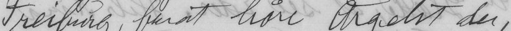Freiburg, forat høre Argelot der,
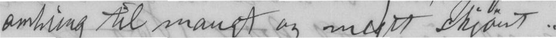omkring til mangt og meget skjønt.
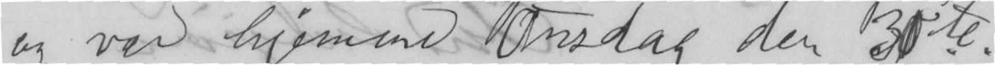og var hjemme Onsdag den 30te.
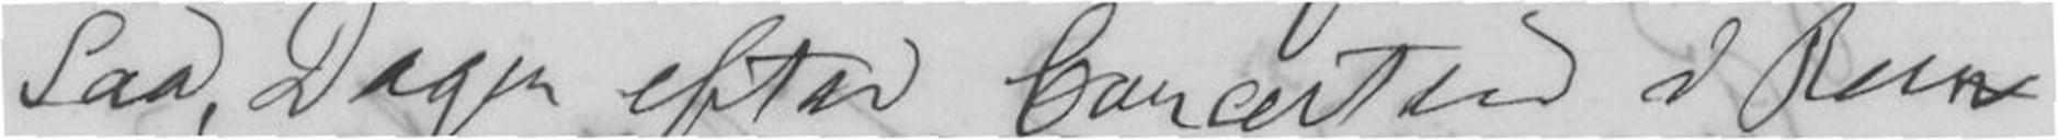Saa, Dagen efter Concerten i Bern
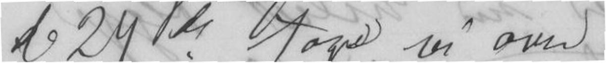d 24de toger vi over
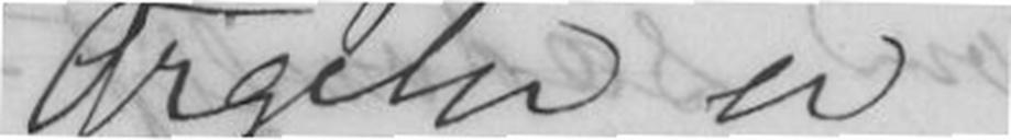Orgeler er
